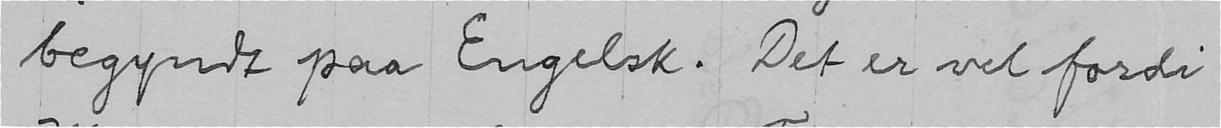begyndt paa Engelsk. Det er vel fordi
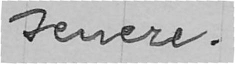senere.
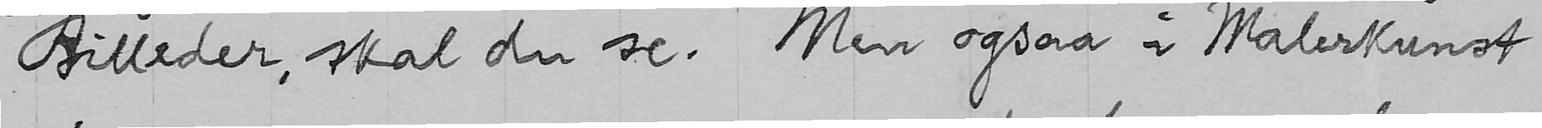Billeder, skal du se. Men ogsaa i Malerkunst
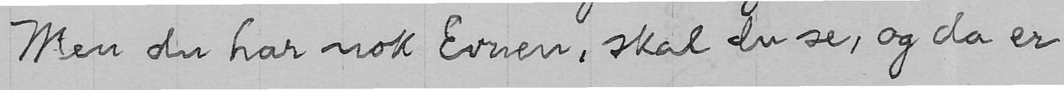Men du har nok Evnen, skal du se, og da er
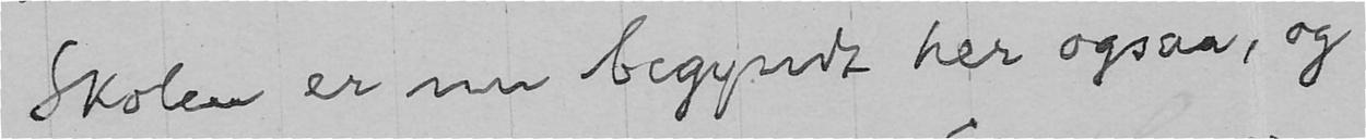Skolen er nu begyndt her ogsaa, og
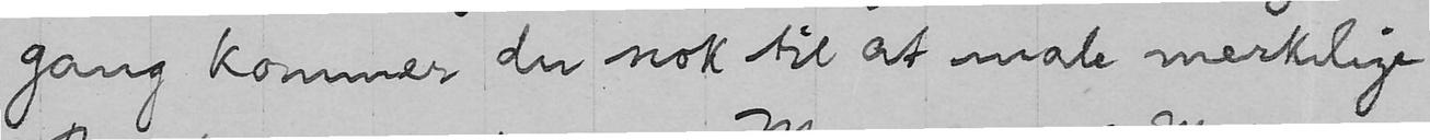gang kommer du nok til at male merkelige
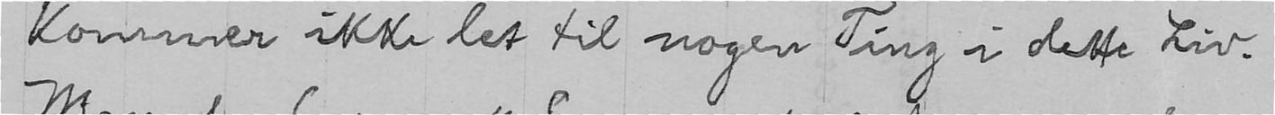kommer ikke let til nogen Ting i dette Liv.
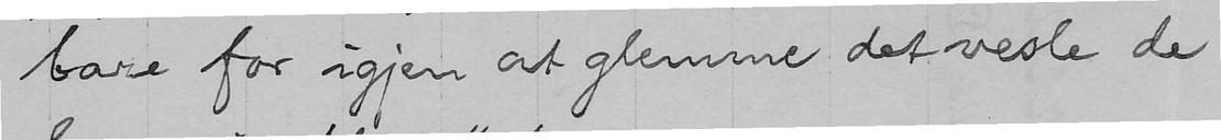bare for igjen at glemme det vesle de
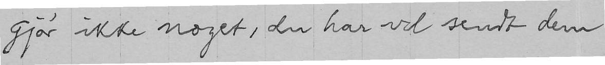gjør ikke noget, du har vel sendt dem
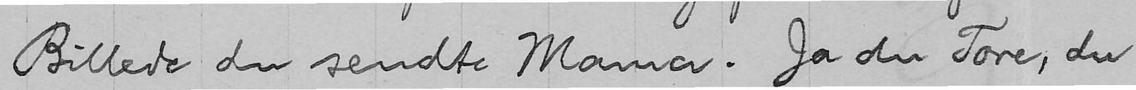Billede du sendte Mama. Ja du Tore, du
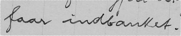faar indbanket.
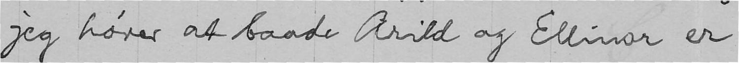jeg hører at baade Arild og Ellinor er
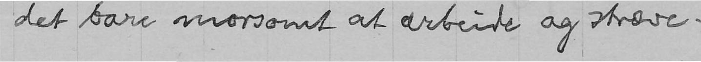det bare morsomt at arbeide og stræve.
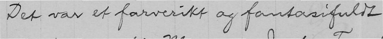Det var et farverikt og fantasifuldt
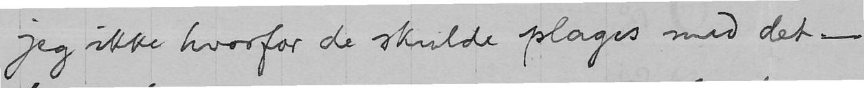jeg ikke hvorfor de skulde plages med det -
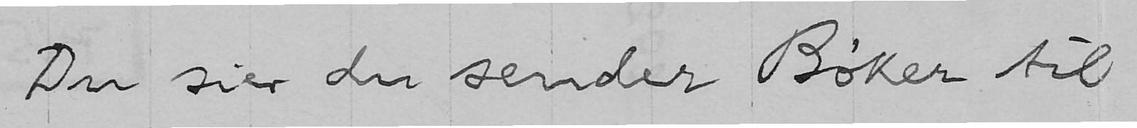Du sier du sender Bøker til
